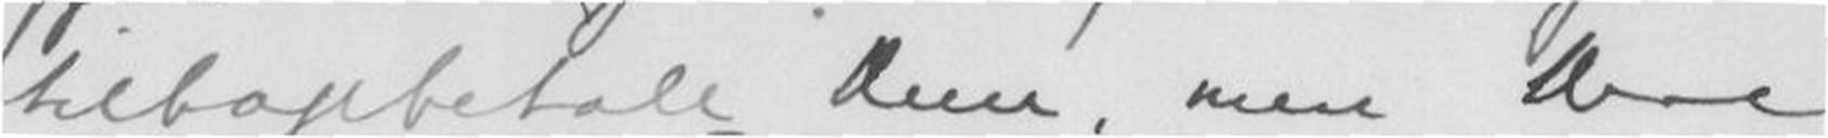tilbagebetale Dem, men Deres
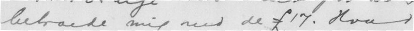betroede mig med de £17. Hvad
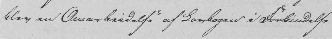blev en Omarbeidelse af Lovbogen i Forbindelse
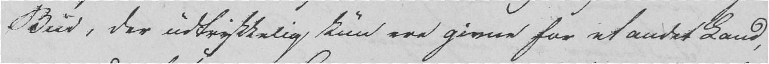Bud, der udtrykkelig kun ere givne for et andet Land,
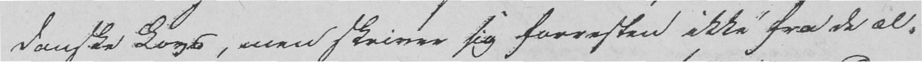danske Lovs, men skriver sig forresten ikke fra de æl-
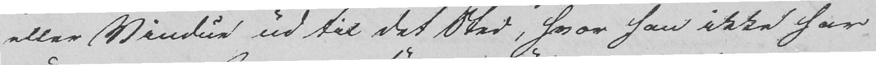eller Vindue ud til det Sted, hvor han ikke har
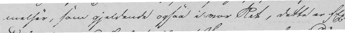melser, som gjeldende ogsaa i vor Ret, dette er f. Ex.
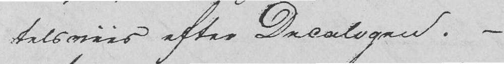telsviis efter Decalogen. -
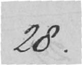28.
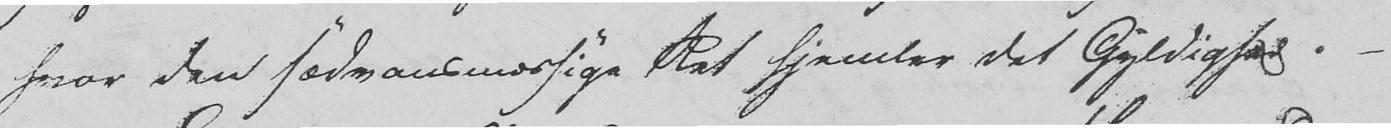hvor den sædvansmæssige Ret hjemler det Gyldighed. -
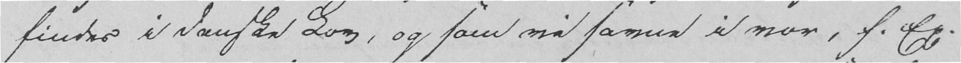findes i danske Lov, og som vi savne i vor, f. Ex.
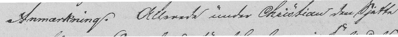Anmærkning. Allerede under Christian den Sjette
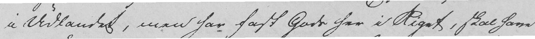i Udlandet, men har fast Gods her i Riget, skal have
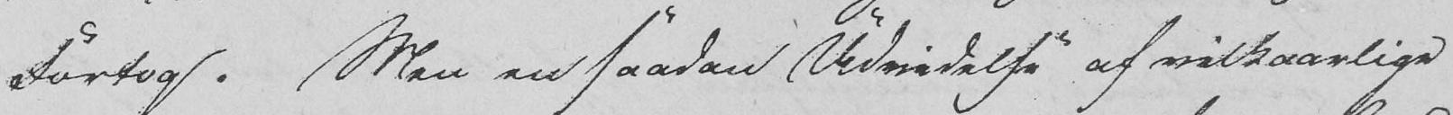Fortog. Men en saadan Udvidelse af vilkaarlige
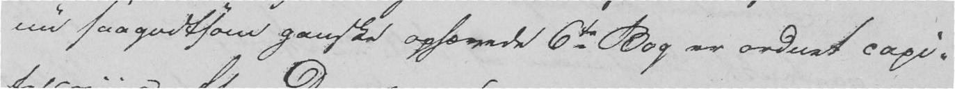nu saagodtsom ganske ophævede 6te Bog er ordnet capi-
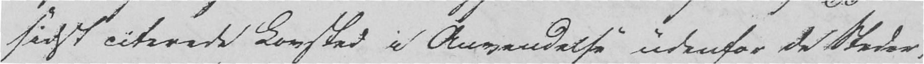sidst citerede Lovsted i Anvendelse udenfor de Steder,
